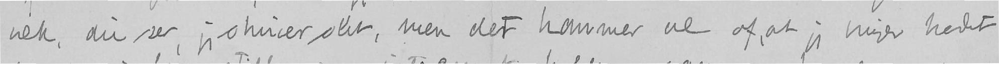vek, du ser, jeg skriver slet, men det kommer vel af, at jeg bruger hodet
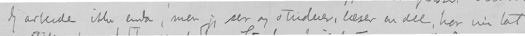Jeg arbeide ikke enda, men jeg ser og studerer, læser en del, har nu tat
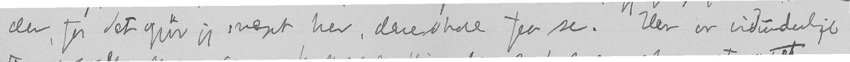der, for det gjør jeg meget her, dere skal faa se. Her er vidunderlige
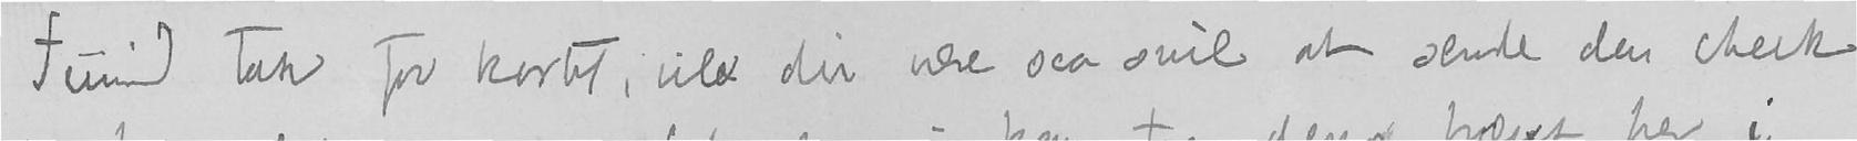Tusind tak for kortet, vil du være saa snil at sende den check
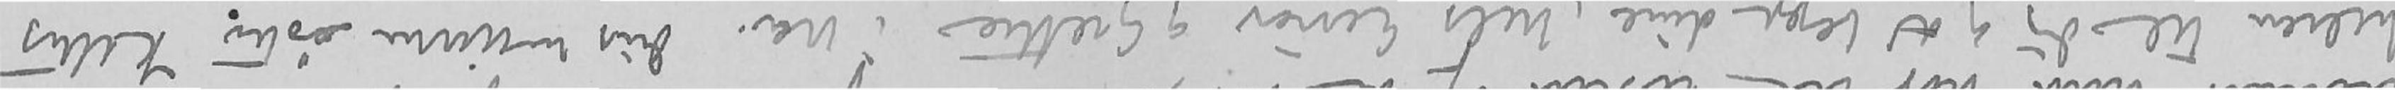hilsen til dig og al begge dine, hils Einar og Grethe i . Din hengivne søster Kitty
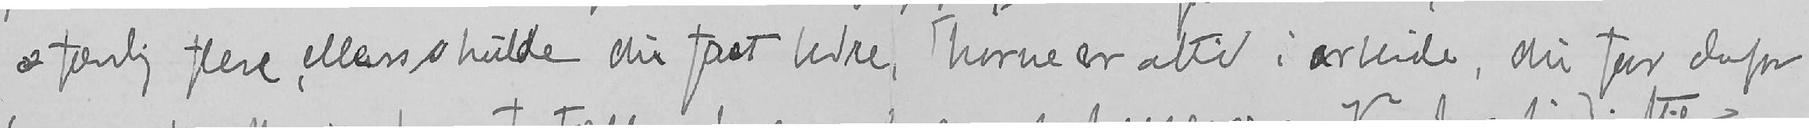færdig flere, ellers skulde du faat bedre, Thorne er altid i arbeide, du faar derfor
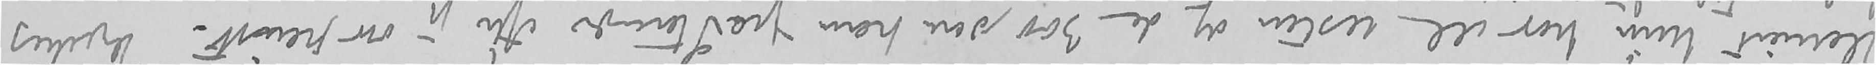Harriet hun har vel resten af de 300 som kom fra Stavanger efter jeg var reist. Hjertelig
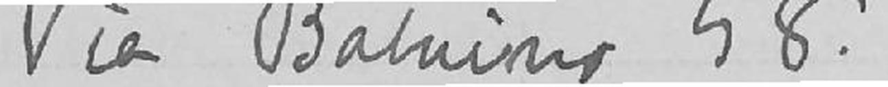Via Babuino 58.
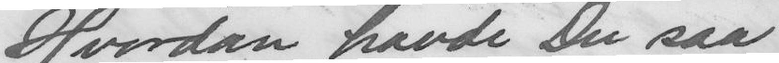Hvordan havde Du saa
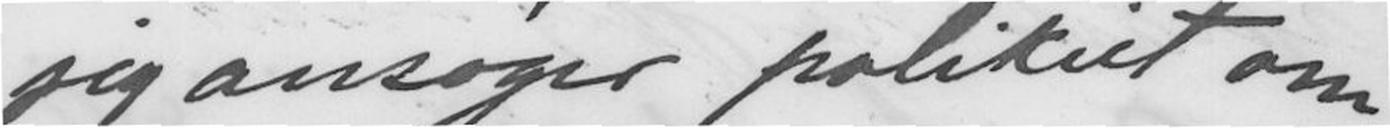jeg ansøger politiet om
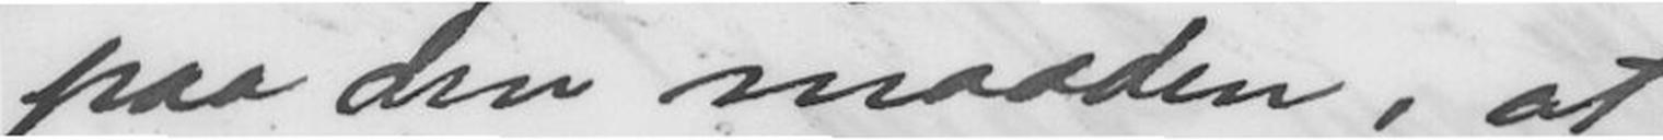paa den maaden, aat
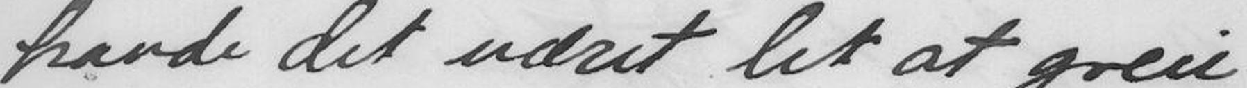havde det været let at greie
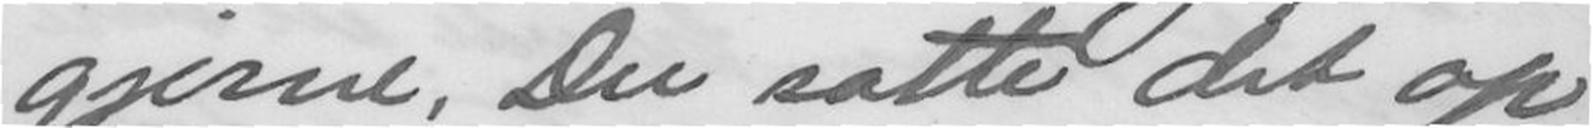gjerne, Du satte det op
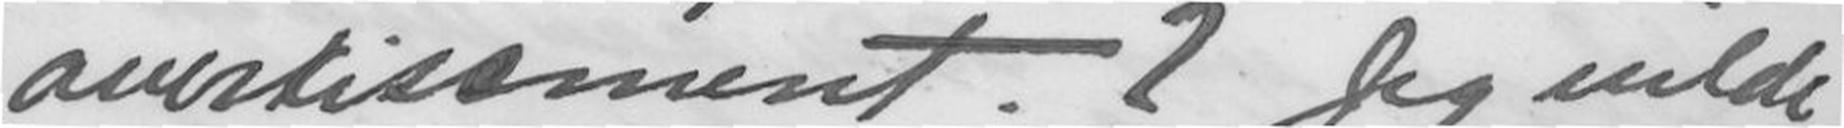avertisement? Jeg vilde
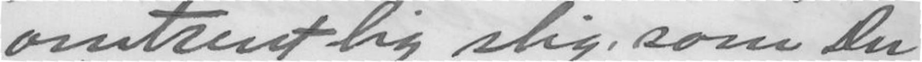omtrentlig slig, som Du
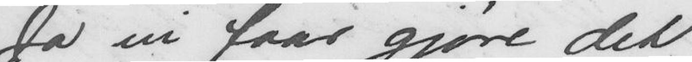Ja vi faar gjøre det
